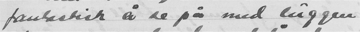fantastisk å se på med luggen
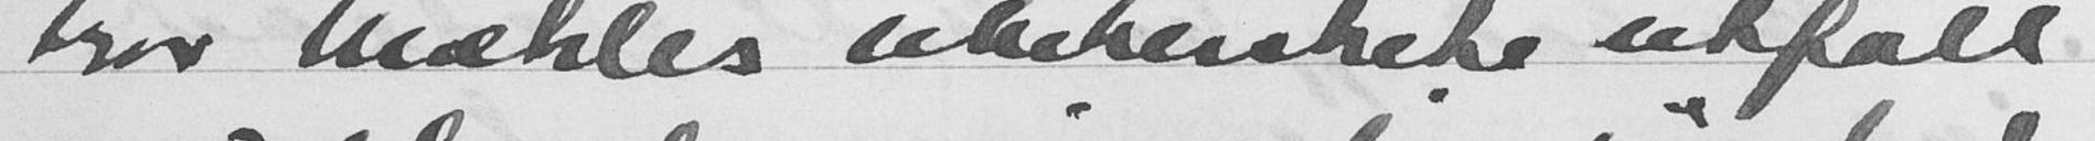tror Mæhles ubeherskete utfall
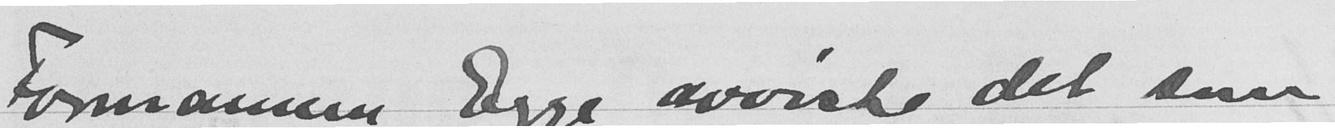Formann Egge avviste det som
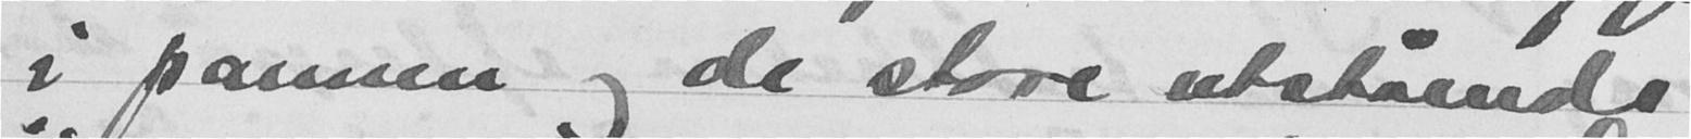i pannen og de store utstående
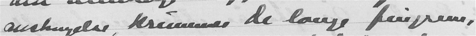anstrengelse, krummer de lange fingrene,
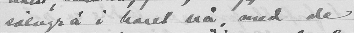sølvgrå i håret nå, med de
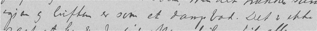igjen og luften er som et dampbad. Det er ikke
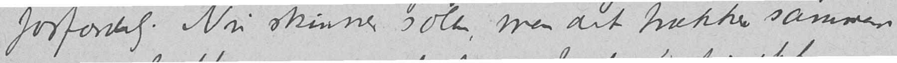forfærdelig. Nu skinner solen, men det trækker sammen
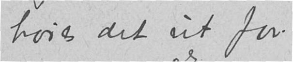høres det ut for.
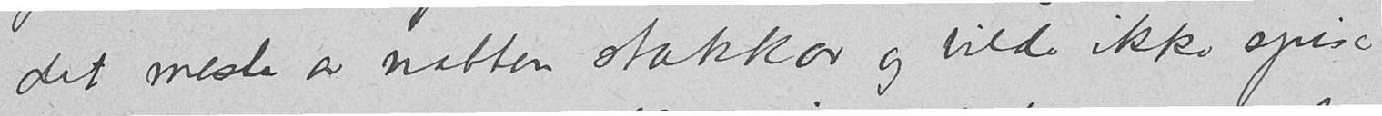det meste av natten stakkar og vilde ikke spise
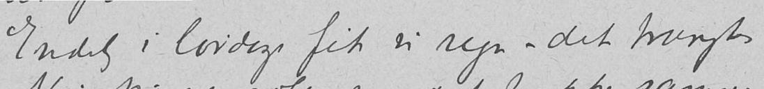Endelig i lørdags fik vi regn – det trængtes
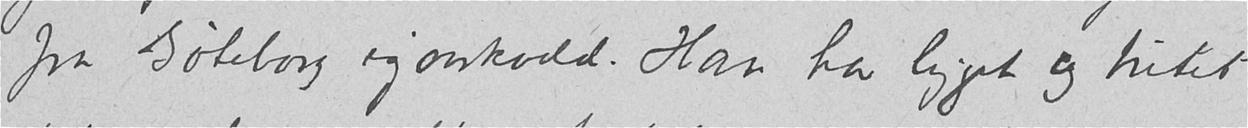fra Gøteborg igaarkveld. Han har ligget og tutet
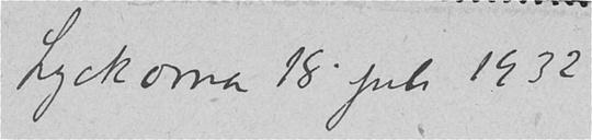Lyckoma 18. juli 1932
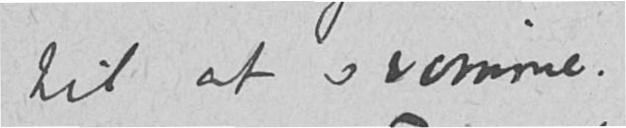til at svømme.
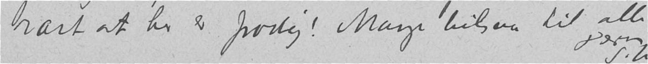rart at her er frodig! Mange hilsener til alle
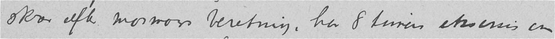skrev efter mormors beretning, har 8 timers eksersis om
